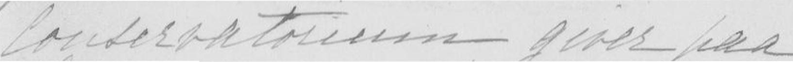conservatorium giver paa
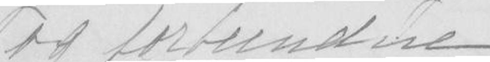og forbundne
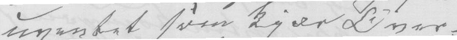uventet som kjær Over-
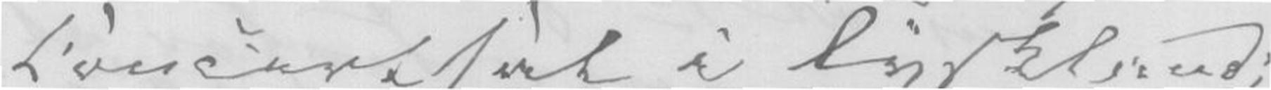Concertsal i Tyskland;
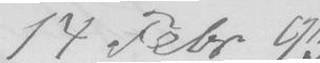14 Febr 93
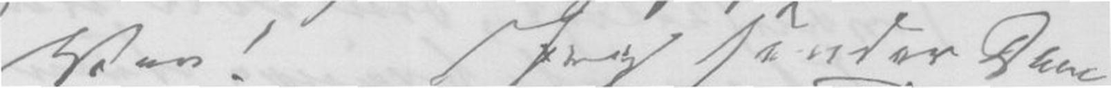Ven!Jeg sender Dem
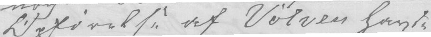Opførelse af Vølven havde
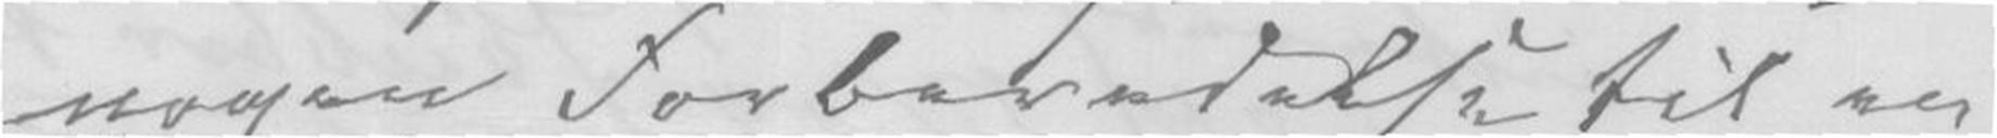nogen Forberedelse til en
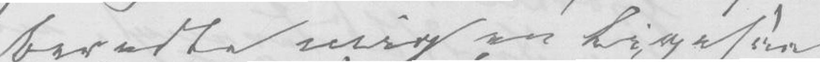beredte mig om ligesaa
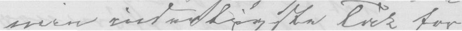min inderligste Tak for
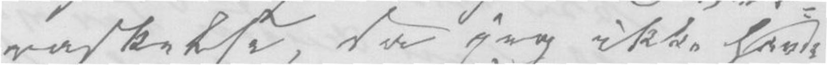raskelse, da jeg ikke havde
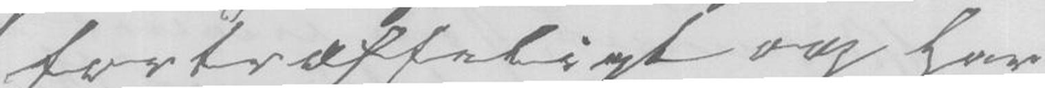fortræffeligt og har
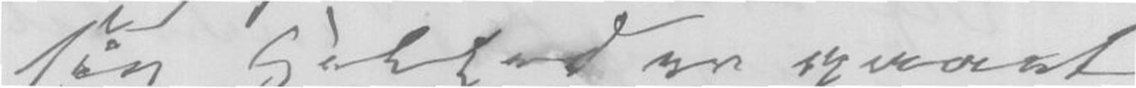sin Helhed er gaaet
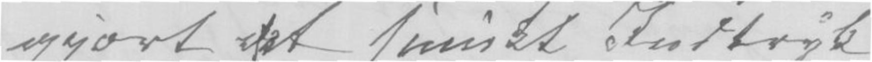gjort et friskt Indtryk
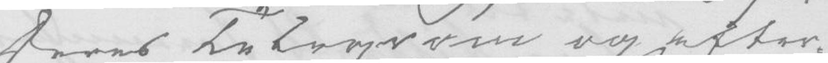Deres Telegram og efte-
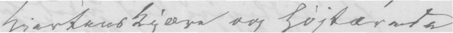Hjertenskjære og højtærede
